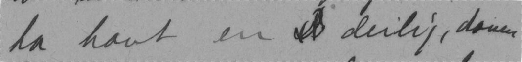ha havt en  deilig, doven
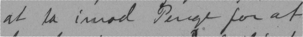at ta imod Penge for at
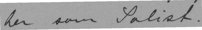her som Solist.
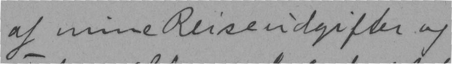af mine Reiseudgifter og
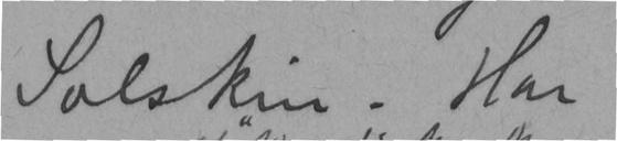Solskin. Har
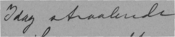Idag straalende
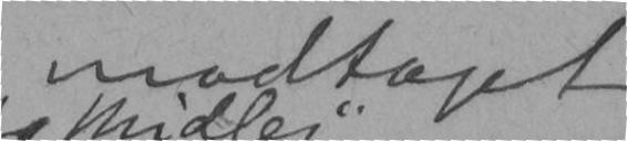modtaget
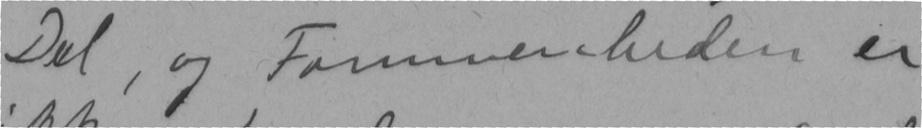Del, og Formuenheden er
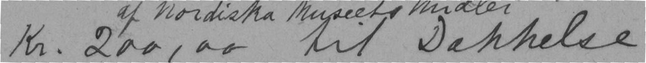Kr. 200,00 til Dækkelse
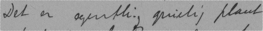Det er egentlig gruelig flaut
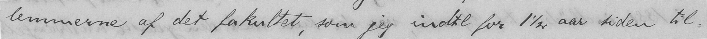lemmerne af det fakultet, som jeg indtil for 1 1/2 aar siden til-
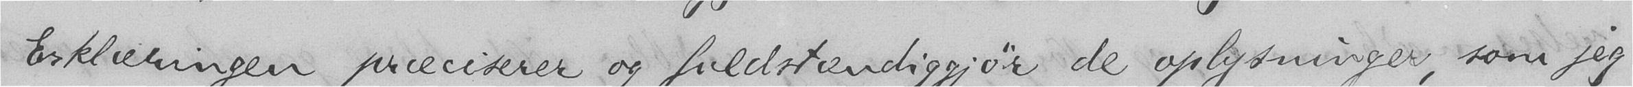Erklæringen præciserer og fuldstændiggjør de oplysninger, som jeg
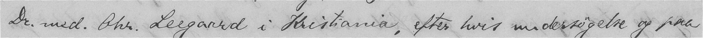Dr. med. Chr. Leegaard i Kristiania, efter hvis undersøgelse og paa
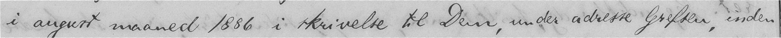i august maaned 1886 i skrivelse til Dem, under adresse Grefsen, inden
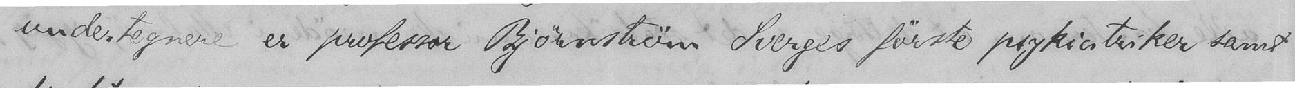undertegnere er professor Björnström Sverges første psykiatriker samt
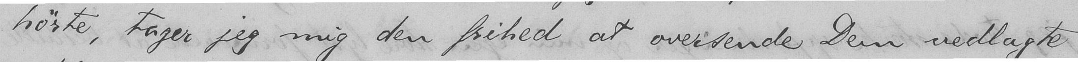hørte, tager jeg mig den frihed at oversende Dem vedlagte
